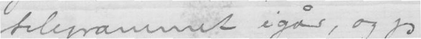telegrammet igår, og jeg
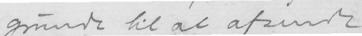grunde til at afrunde
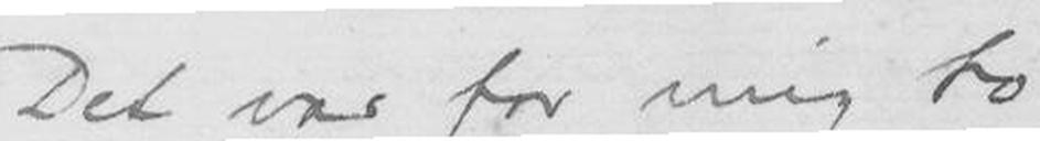Det var for mig to
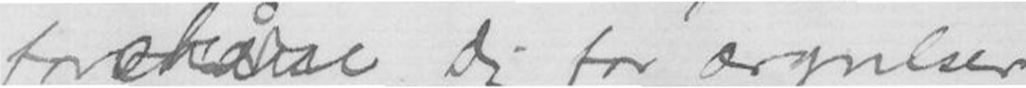forskåne dig for ærgelser
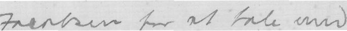Jacobsen for at tale med
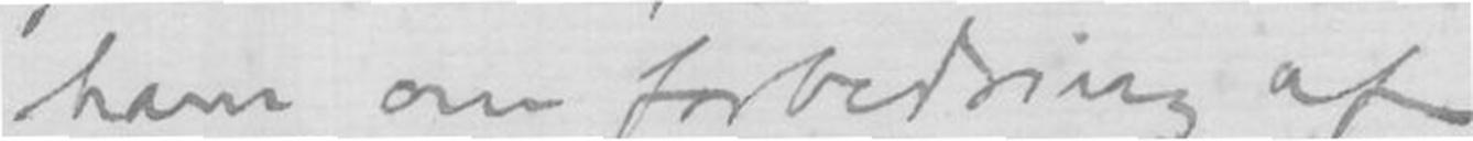ham om forbedring af
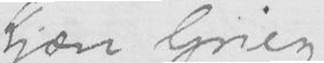Kjære Grieg!
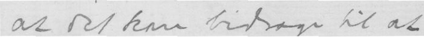at det kan bidrage til at
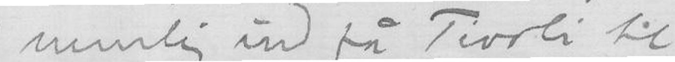nemlig ind på Tivoli til
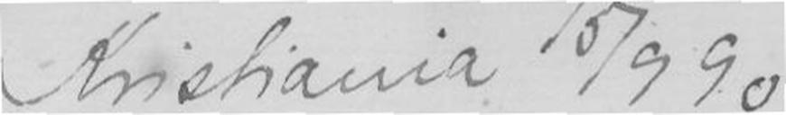Kristianina 15/9 90
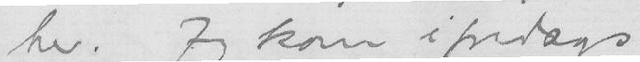her. Jeg kom ifredags
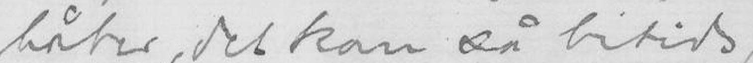håber, det kom så bitids
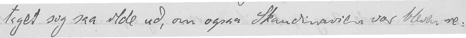taget sig saa ilde ud, om ogsaa Skandinavien var bleven re-
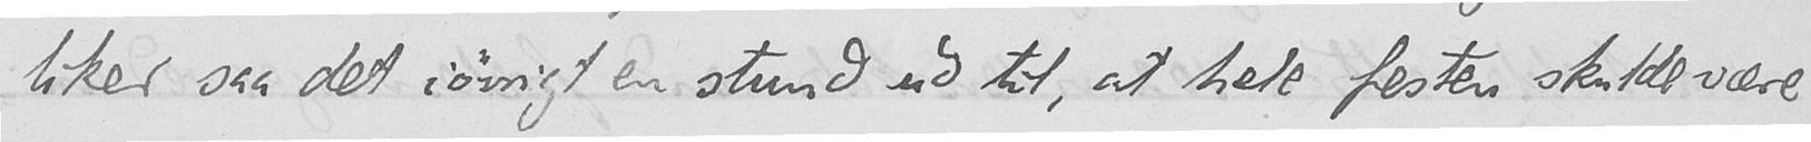liker saa det iøvrigt en stund ud til, at hele festen skulde være
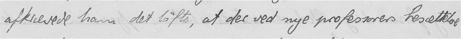afkrævede ham det løfte, at der ved nye professorers besættelse
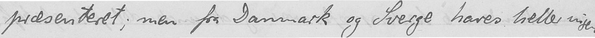præsenteret; men fra Danmark og Sverge haves heller ingen
